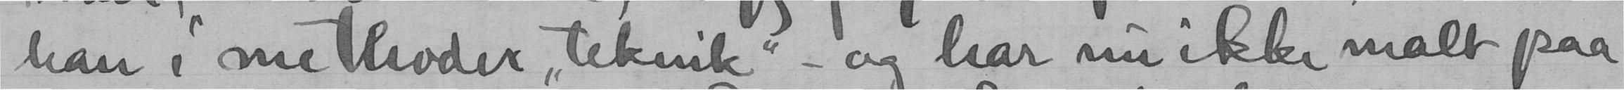han i methoder "teknik" - og har nu ikke malt paa
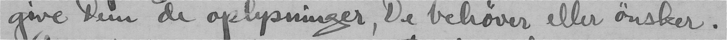give Dem de oplysninger, De behøver eller ønsker.
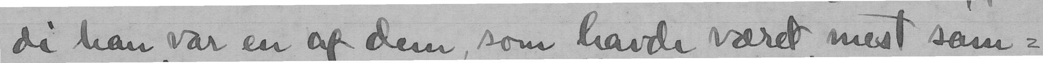di han var en af dem, som havde været mest sam-
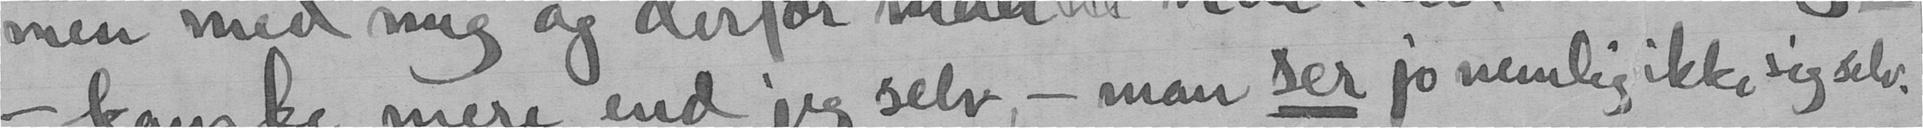- kanske mere end jeg selv - man ser jo nemlig ikke sig selv.
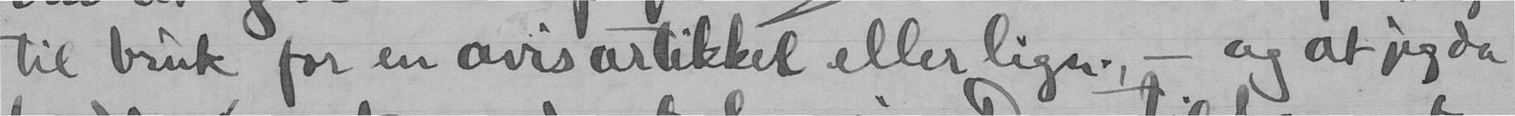til bruk for en avisartikket eller lign, - og at jeg da
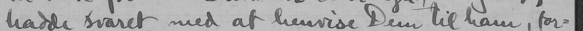hadde svaret med at henvise Dem til ham, for-
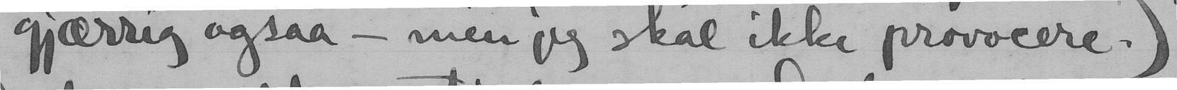gjærrig ogsaa - men jeg skal ikke provocere.)
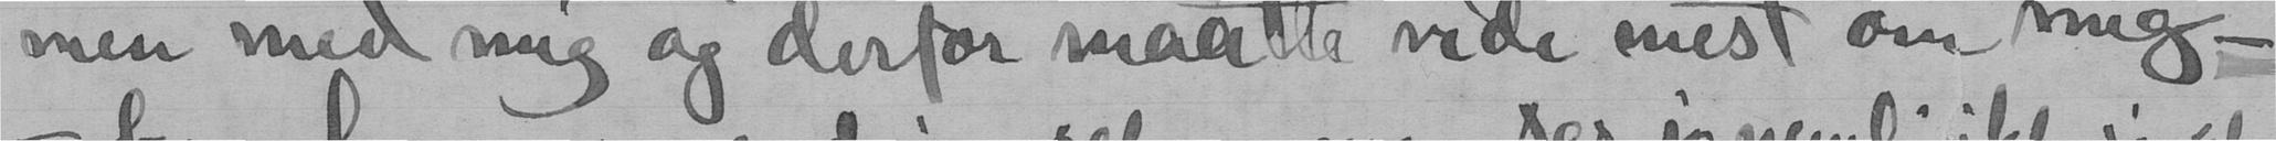men med mig og derfor maatte vide mest om mig -
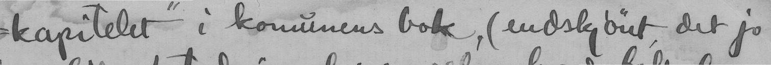kapitelet" i komunens bok, (endskjønt det jo
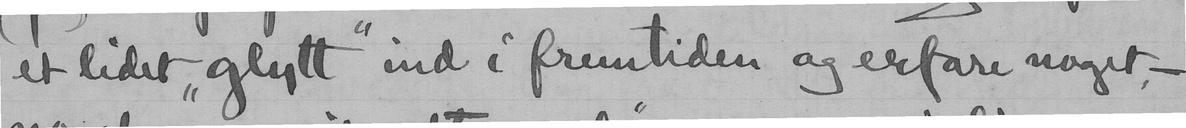et lidet "glytt" ind i fremtiden og enfare noget, -
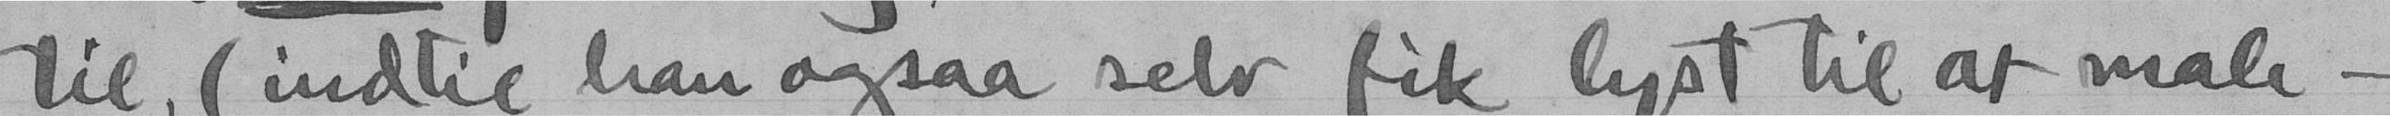til, (indtil han ogsaa selv fik lyst til at male-
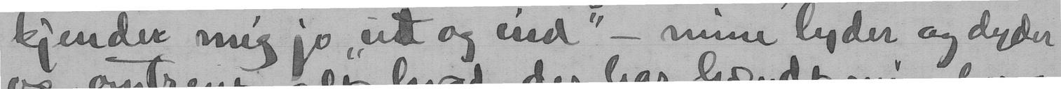kjender mig jo "ud og ind" - mine lyder og dyder
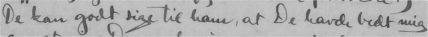De kan godt sige til ham, at De havde bedt mig
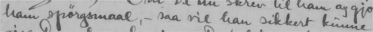ham spørgsmaal, - saa vil han sikkert kunne
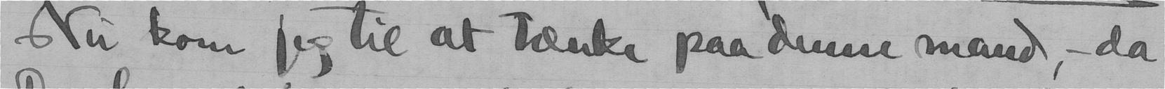Nu kom jeg til at tænke paa denne mand, - da
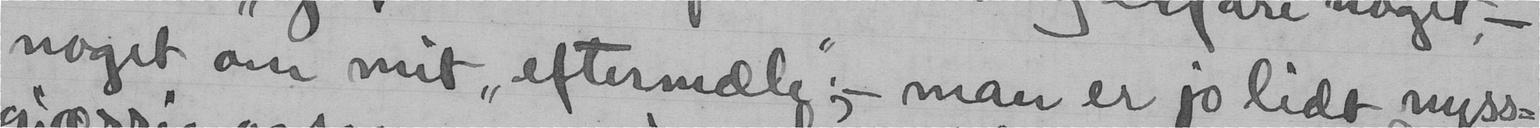noget om mit "eftermæle"; - man er jo lidt nyss-
